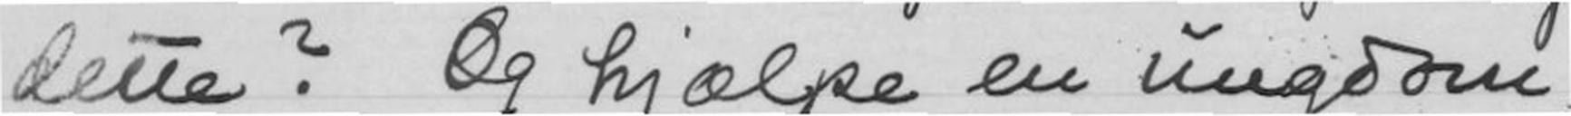dette? Og hjælpe en ungdom
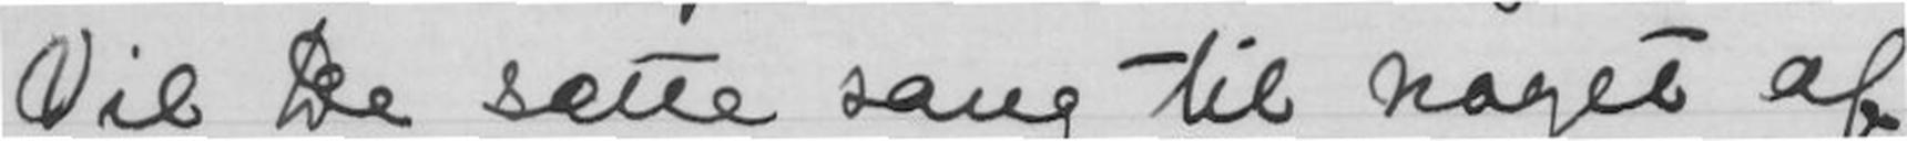Vil De sætte sang til noget af
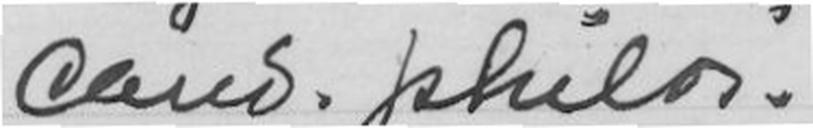Cand. philos.
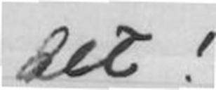det!
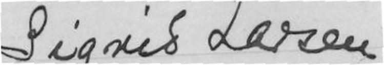Sigrid Larsen.
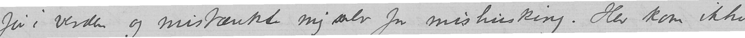før i verden og mistænkte mig selv for mishusking. Her kom ikke
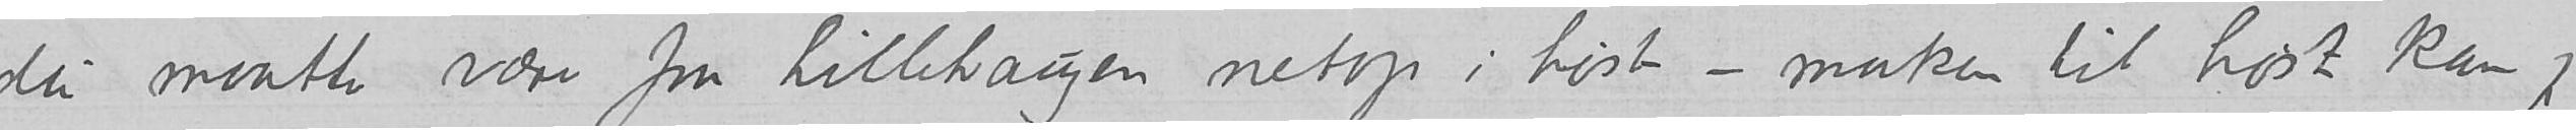du maatte være fra Lillehaugen netop i høst – maken til høst
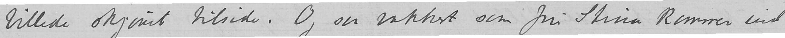billede skjøvet tilside. Og saa vakkert som fru Stina kommer ind
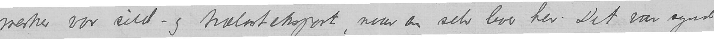merker vor sild- og trælasteksport, naar enselv lever her. Det var synd
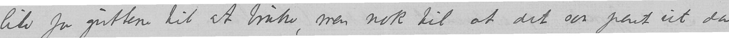lite for guttene til at bruke, men nok til at det saa pent ut da
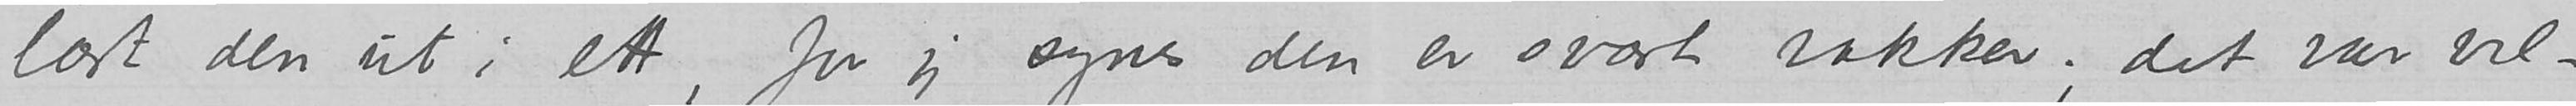læst den ut i ett, for jeg syns den er svært vakker; det var vel
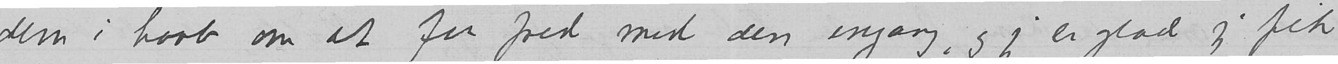den i haab om at faa fred med den engang, og jeg er glad jeg fik
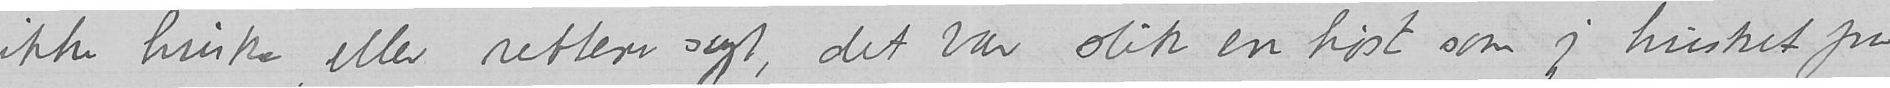kan jeg ikke huske, eller rettere sagt, det var slik en høst som jeg husket fra
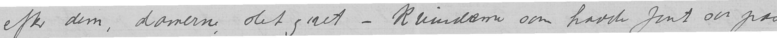efter dem, damerne, slet og ret – kvinderne som hadde faat saa pas
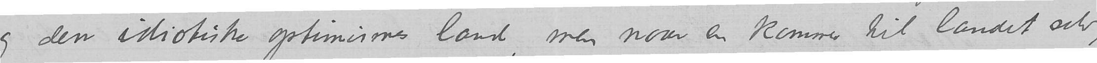og den idiotiske optimismes land, men naar en kommer til landet selv,
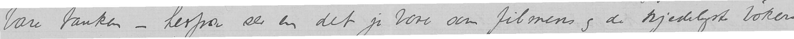bare tanken – herfra ser en det jo bare som filmens og de kjedelige bøkers
